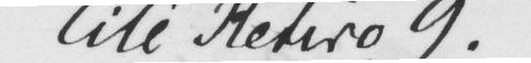Cité Retiro 9.
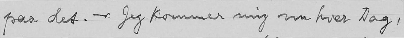paa det. - Jeg kommer mig nu hver Dag,
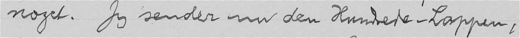noget. Jeg sender nu den Hundrede-Lappen,
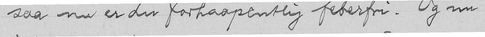saa nu er du forhaapentlig feberfri. Og nu
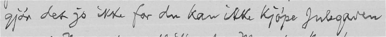gjør det jo ikke for du kan ikke kjøpe Julegaven
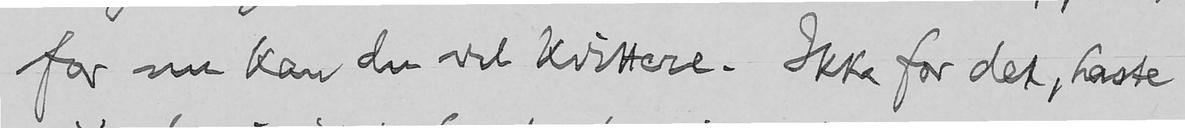for nu kan du vel kvittere. Ikke for det, haste
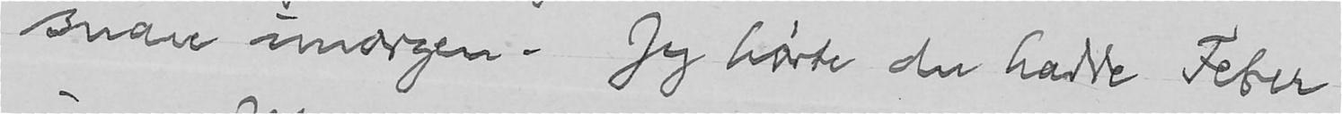snau imorgen. Jeg hørte du hadde Feber
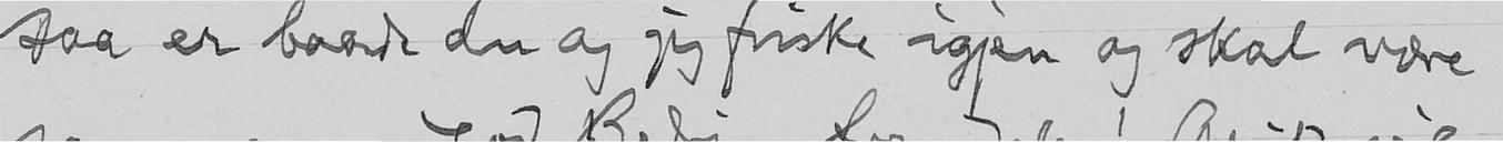saa er baade du og jeg friske igjen og skal være
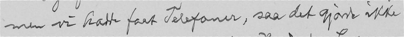men vi hadde faat Telefoner, saa det gjorde ikke
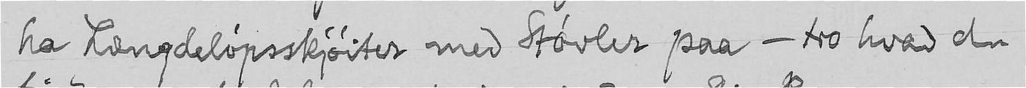ha Længdeløpsskjøiter med Støvler paa - tro hvad du
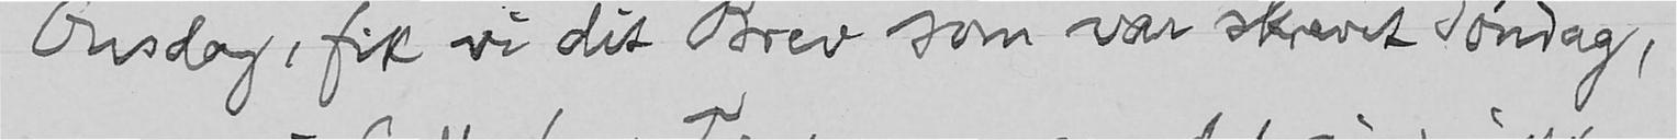Onsdag, fik vi dit Brev som var skrevet Søndag,
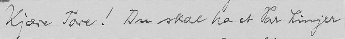Kjære Tore! Du skal ha et Par Linjer
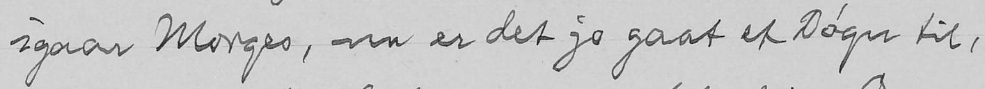igaar Morges, nu er det jo gaat et Døgn til,
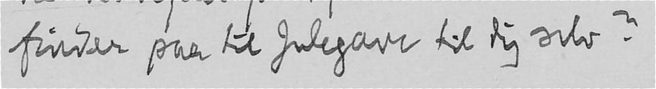finder paa til Julegave til dig selv?
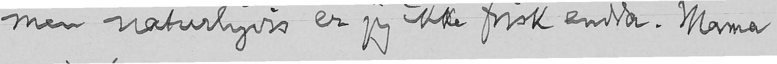men naturligvis er jeg ikke frisk endda. Mama
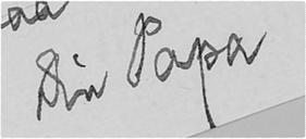Din Papa
